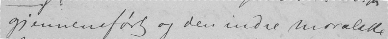gjennemført og den indre moralske
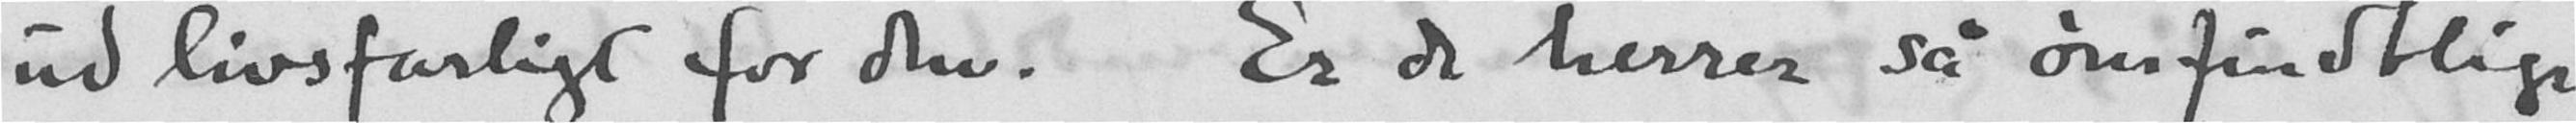ud livsfarligt for den. Er de herrer så ømfiendtlige
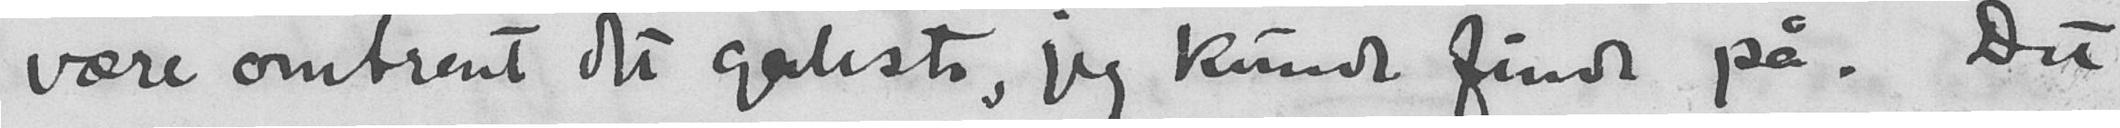være omtrent det galeste, jeg kunde finde på. Det
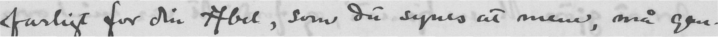farligt for din Abel, som du synes at mene, må gan-
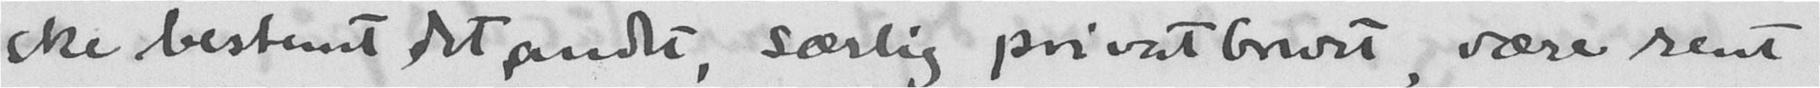ske bestemt det andet, særlig privatbrevet, være rent
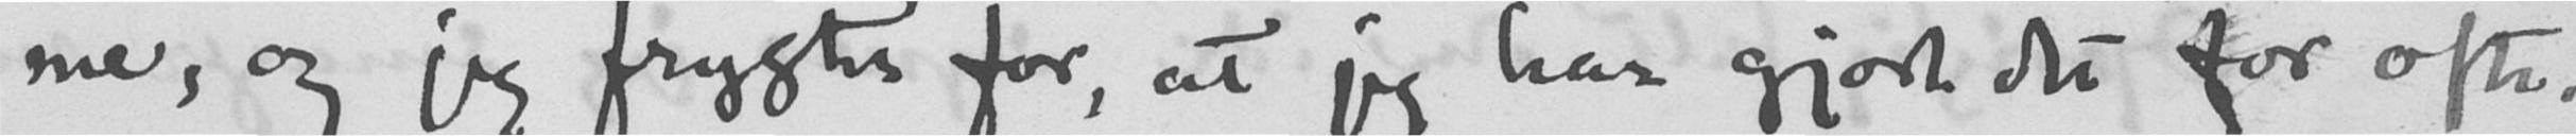me, og jeg frygter for, at jeg har gjort det for ofte.
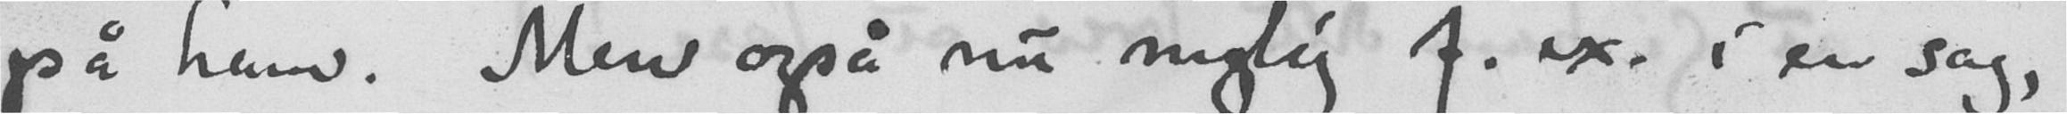på ham. Men også nu nylig f. ex. i en sag,
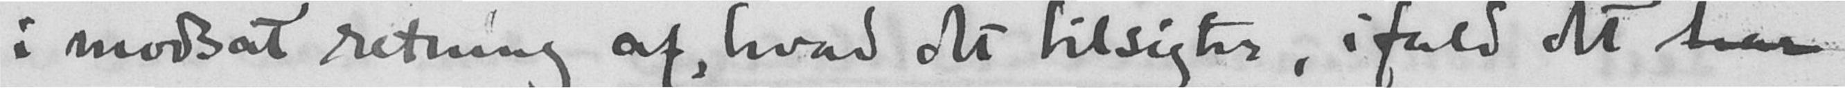i modsat retning af, hvad det tilsigter, ifald det har
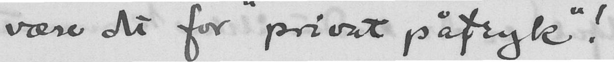være det for "privat påtryk"!
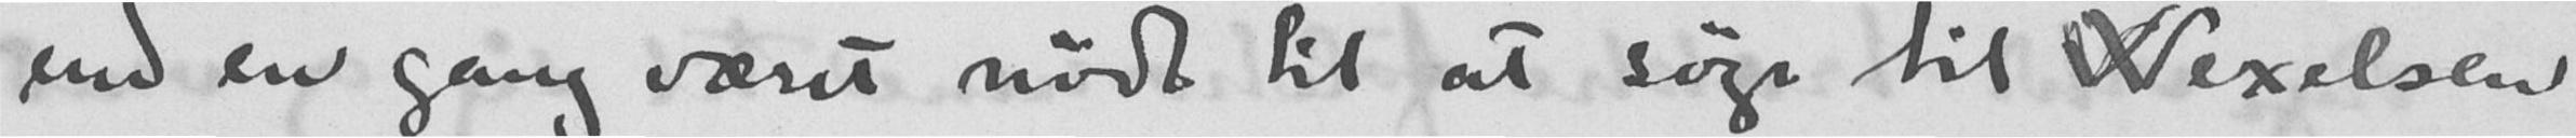end en gang været nødt til at søge til Wexelsen
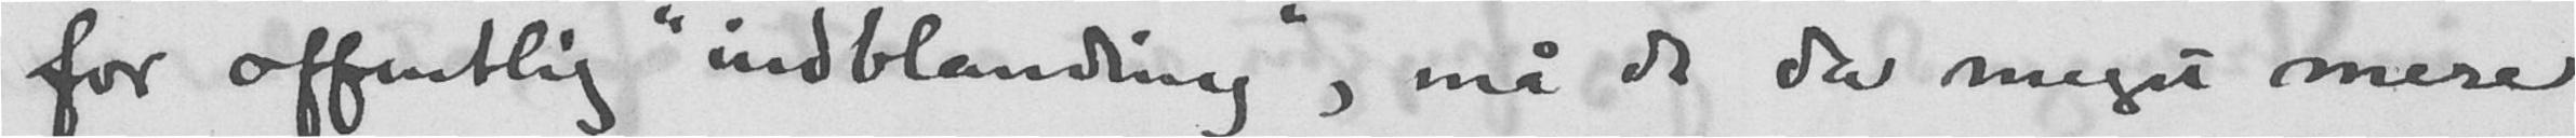for offentlig "indblanding", må de da meget mere
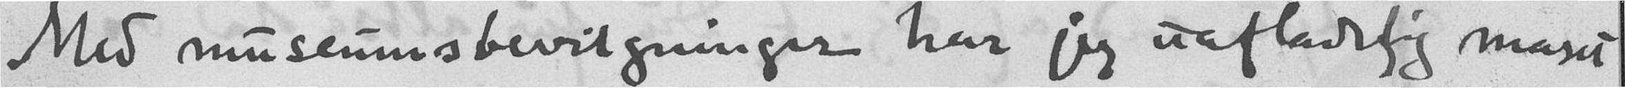Med museumsbevilgninger har jeg uafladelig maset
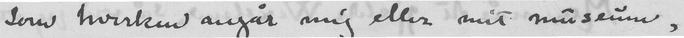som hverken angår mig eller mit museum,
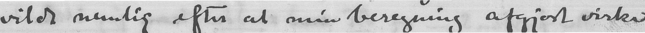vilde nemlig efter al min beregning afgjort virke
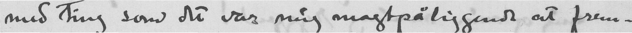med ting som det var mig magtpåliggende at frem-
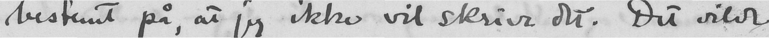bestemt på, at jeg ikke vil skrive det. Det vilde
